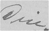Din
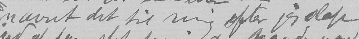nævnt det til mig efter jeg slap
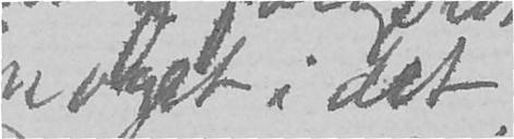noget i det.
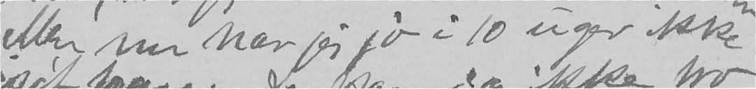Men nu har jeg jo i 10 uger ikke
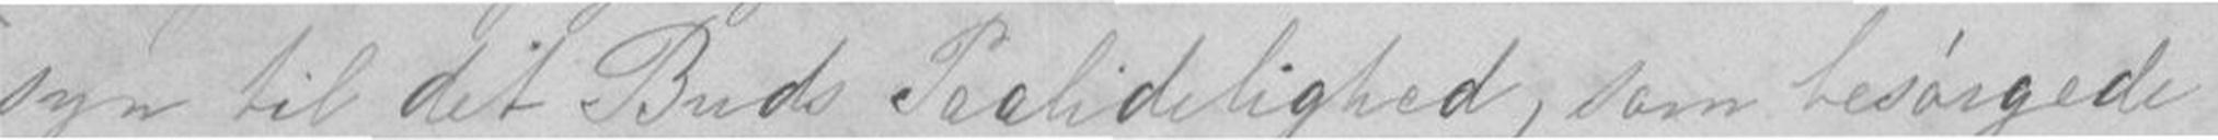syn til det Buds Paalidelighed, som besørgede
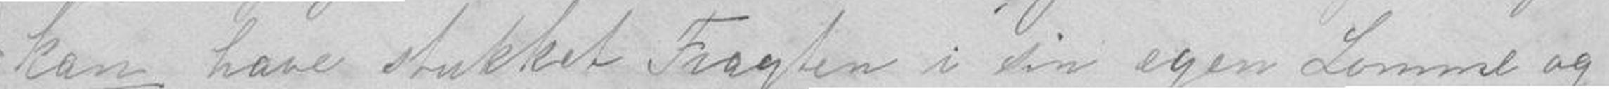kan have stukket Frakten i sin egen lomme og
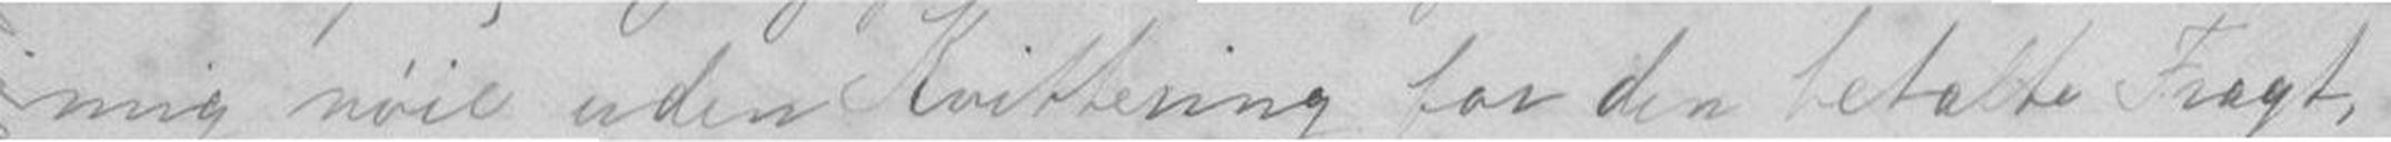mig nøie uden Kvittering for den betalte Fragt,
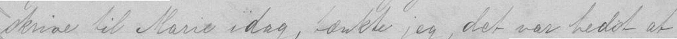skrive til Marie idag, tænkte jeg , det var bedst at
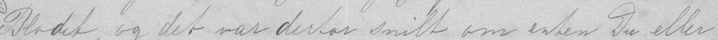Blodet, og det var derfor snilt om enten Du eller
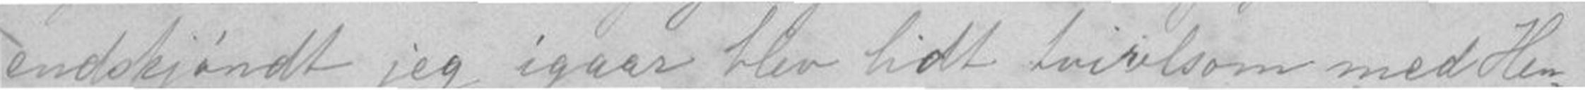endskjøndt jeg igaar bliv lidt tvirlsom med Hen-
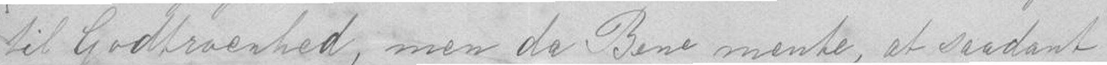til Godtroenhed, men da Bene mente, at saadant
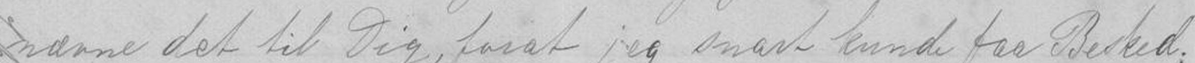nævne det til Dig, forat jeg snart kunde faa Besked;
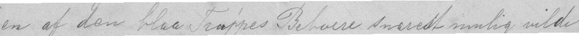en af dem blaa Trappes Beboere snarest mulig vilde
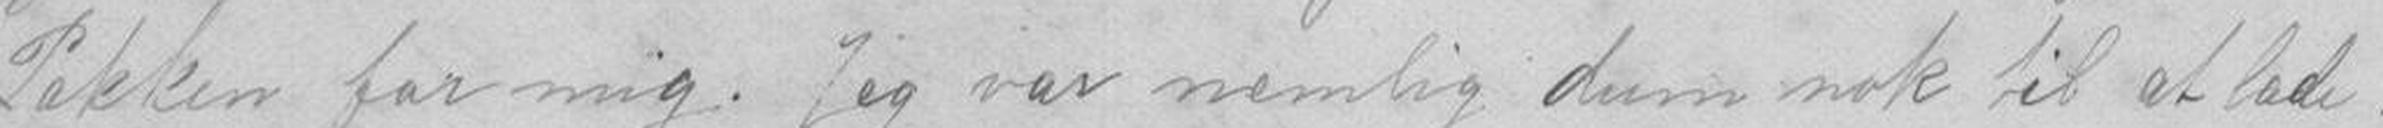Pakken for mig. Jeg var nemlig dum nok til at lade
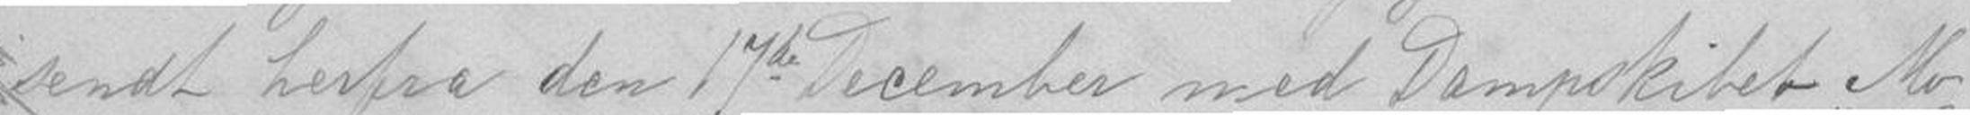sendt herfra den 17de December med Dampskibet. Mo-
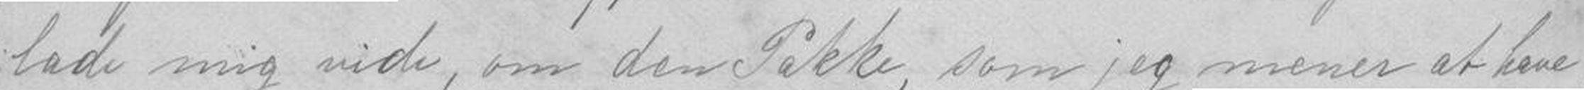lade mig vide, om den Pakke, som jeg mener at have
